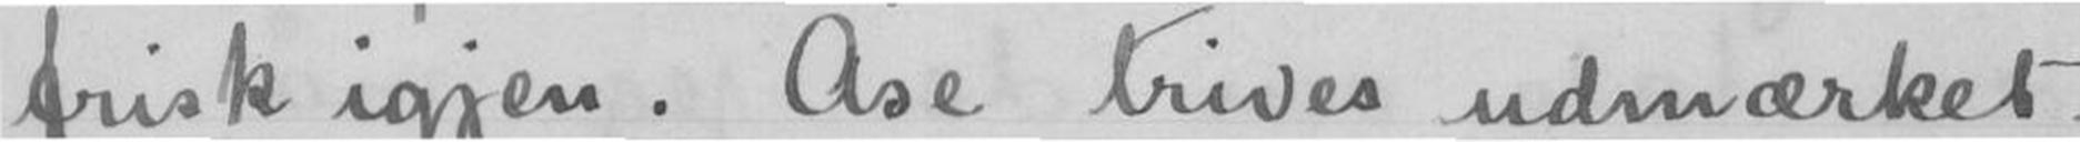frisk igjen. Åse trives udmærket.
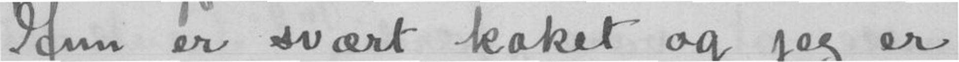Hun er svært koket og jeg er
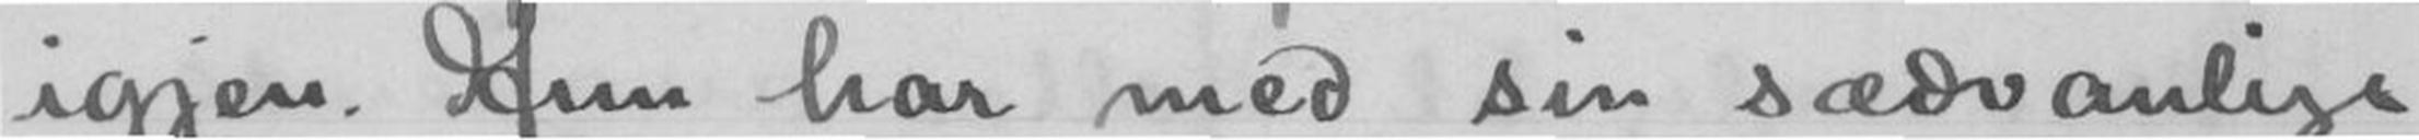igjen. Hun har med sin sædvanlige
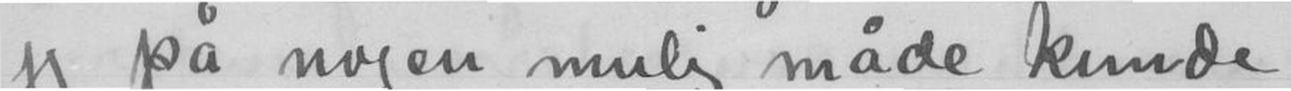jeg på nogen mulig måde kunde
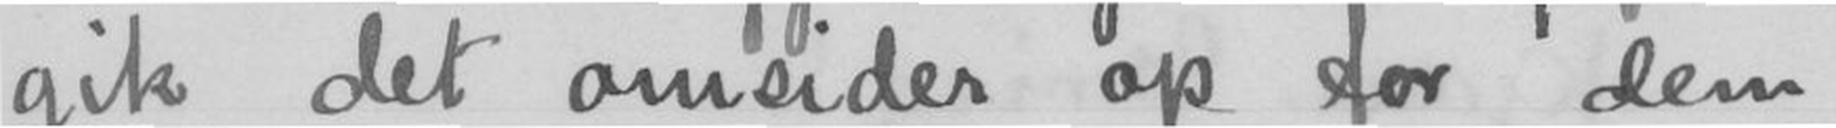gik det omsider op for dem
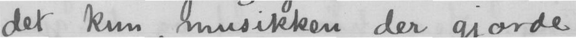der kun musikken der gjorde
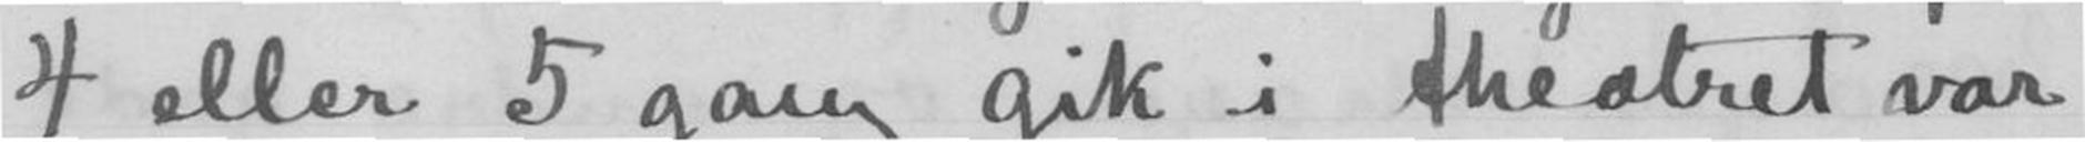4 eller 5 gang gik i theatret var
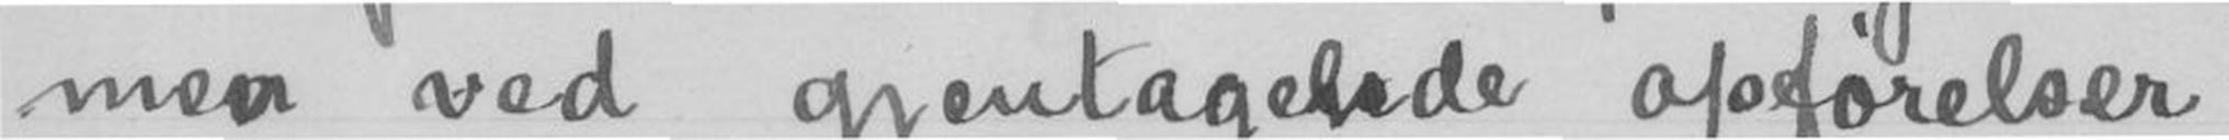men ved gjentagende opførelser
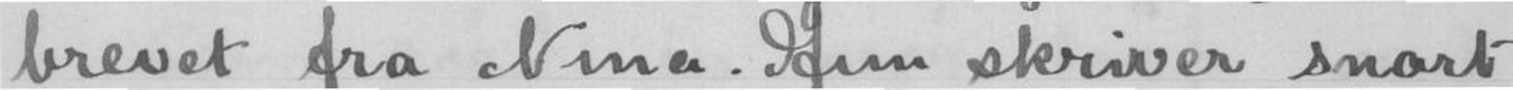brevet fra Nina. Hun skriver snart
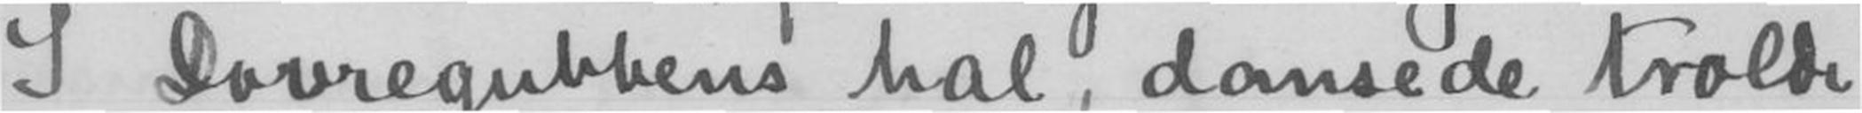I Dovregubbens hal, dansede trolde
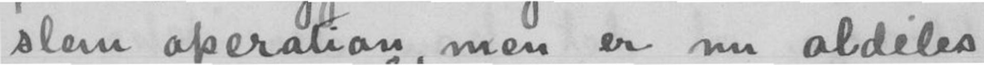slem operation, men er nu aldeles
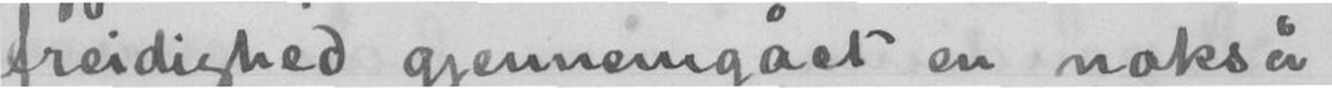freidighed gjennemgået en nokså
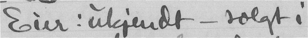Eier: ukjendt - solgt i
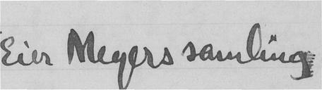Eier Meyers samling
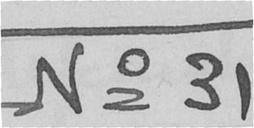No 31
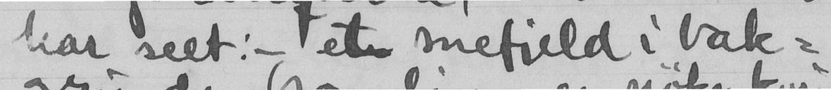har seet : - et snefjeld i bak-
